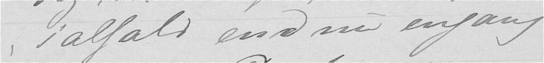ialfald endnu engang
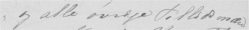og alle øvrige Tillidsmænd
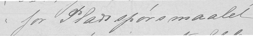for Pladsspørsmaalet
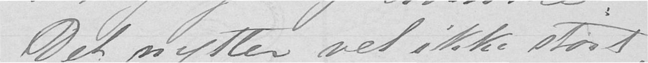Det nytter vel ikke stort,
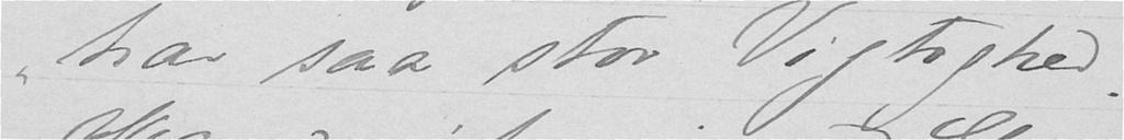har saa stor Vigtighed.
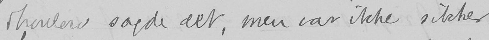Thaulow sagde det, men var ikke sikker.
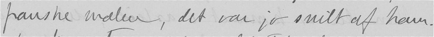franske malere, det var jo snilt af ham.
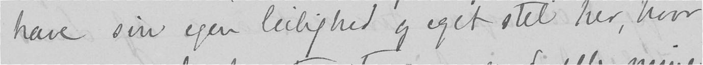have sin egen leilighed og eget stel her, hvor
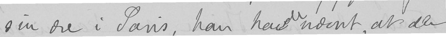sin ære i Paris, han havde nævnt, at der
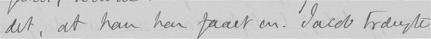det, at han har faaet en. Jacob trængte
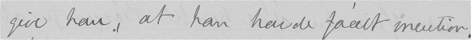give han, at han havde faaet mention.
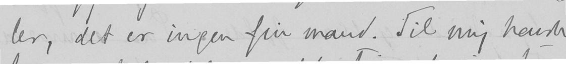ler, det er ingen fin mand. Til mig havde
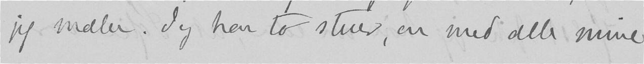jeg maler. Jeg har to stuer, en med alle mine
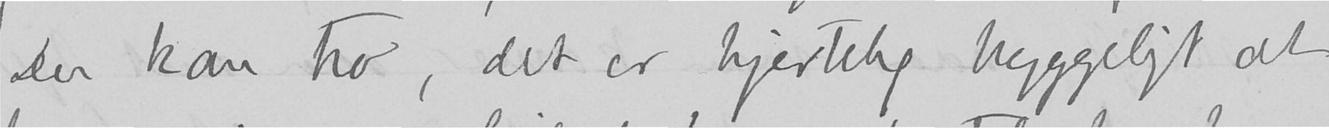Du kan tro, det er hjertelig hyggeligt at
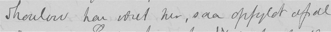Thaulow har været her, saa opfyldt af al
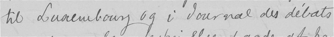til Luxembourg og i Journal des débats
